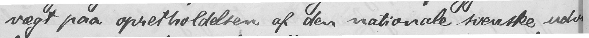vægt paa opretholdelsen af den nationale svenske udvik-
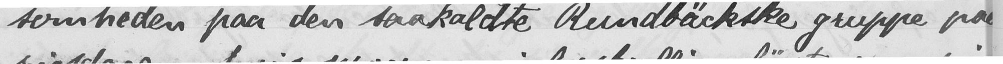somheden paa den saakaldte Rundbäckske gruppe paa
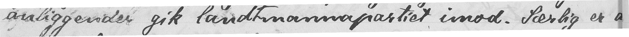anliggender gik landtmannapartiet imod. Særlig er det
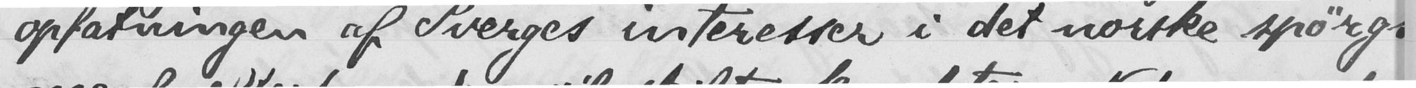opfatningen af Sverges interesser i det norske spørgs-
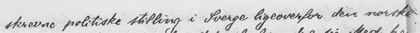skrevne politiske stilling i Sverge ligeoverfor den norske
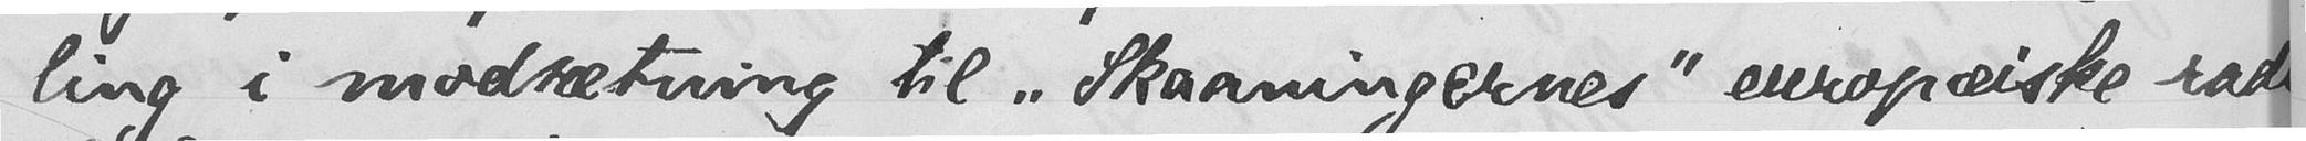ling i modsætning til "Skaaningernes" europæiske radi-
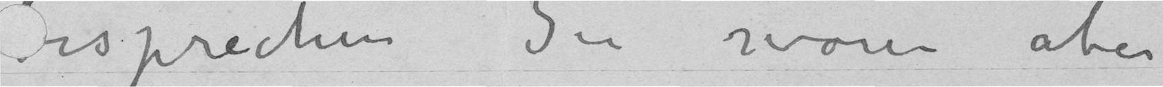besprechen Sie waren aber
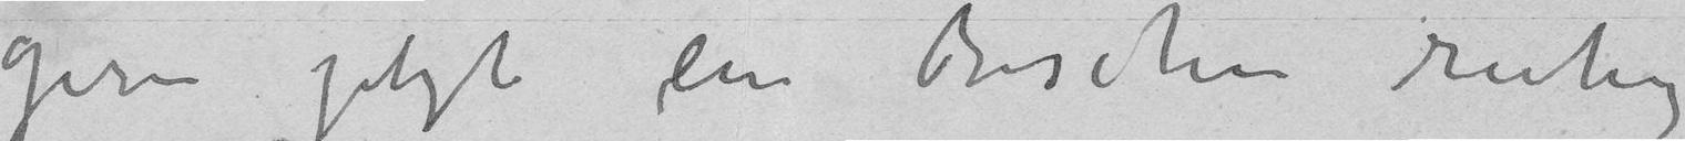gern jetzt ein Bischen ruhig
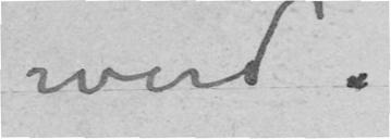wird.
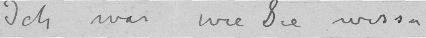Ich war wie Sie wissen
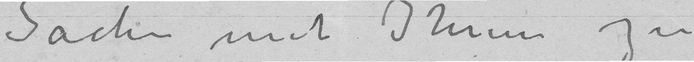Sachen mit Ihnen zu
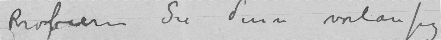Probieren Sie denn vorlaufig
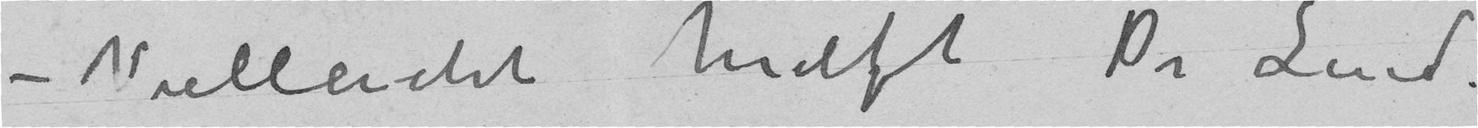– Vielleicht hielft Dr Linde
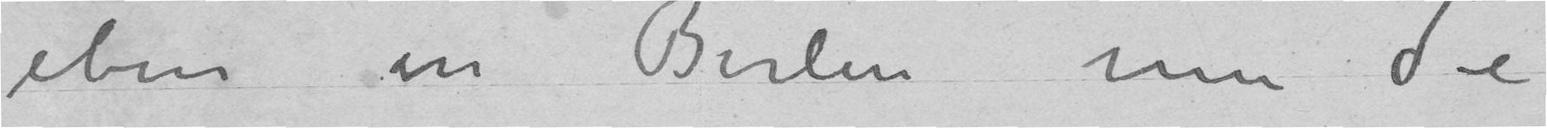eben in Berlin um die
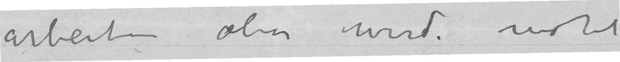arbeiten aber wirde wohl
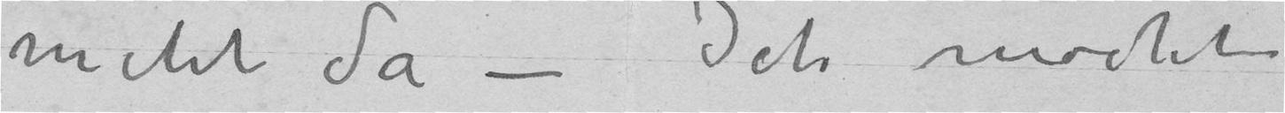nicht da – Ich mochte
